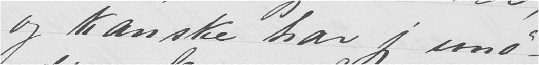og kanske har jeg unø-
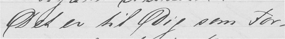Det er til Dig som For-
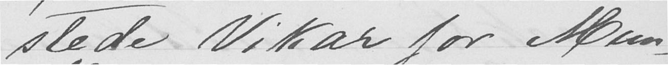stede Vikar for Mun-
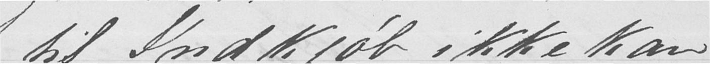til Indkjøb ikke kan
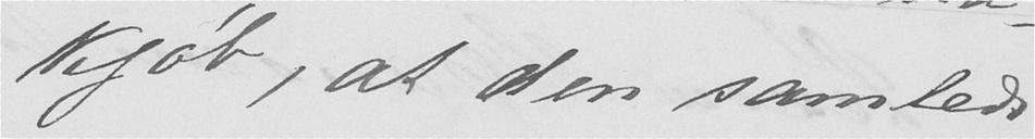kjøb, at den samlede
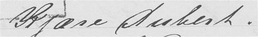Kjære Aubert.
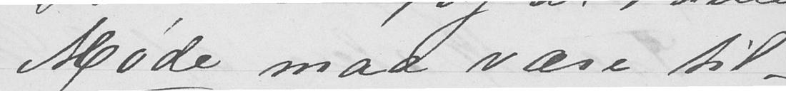Møde maa være til-
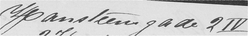Hansteens gade 2 IV
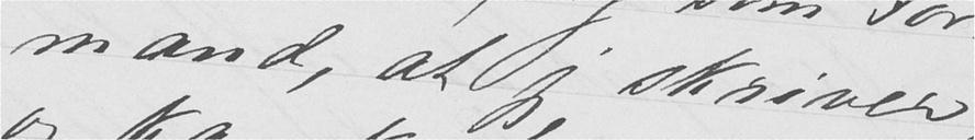mand, at jeg skriver
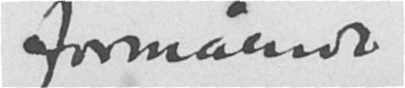formående
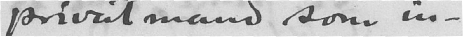privatmand som in-
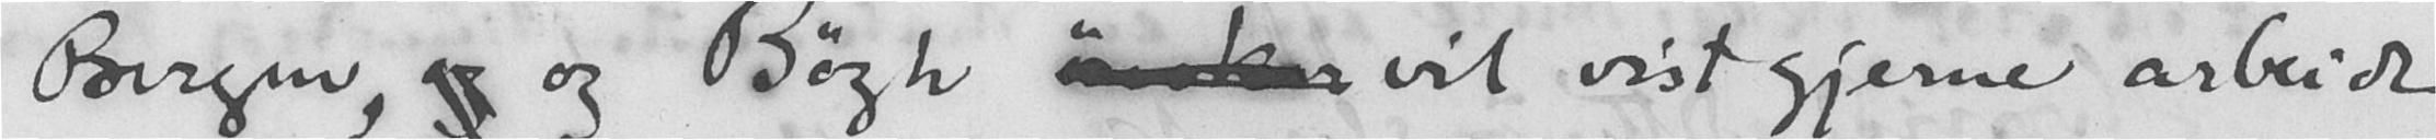Bergen, og og Bøgh ønsker vil vist gjerne arbeide
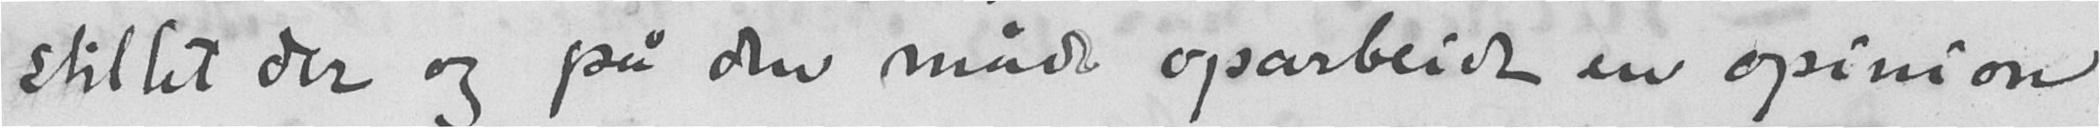stillet der og på den måde oparbeide en opinion
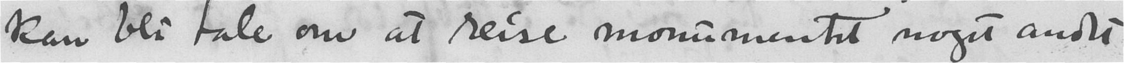kan bli tale om at reise monumentet noget andet
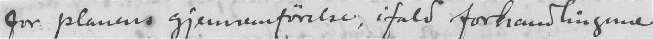for planens gjennemførelse, ifald forhandlingerne
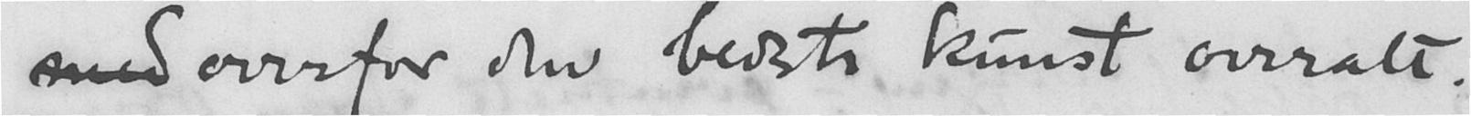med overfor den bedste kunst overalt.
